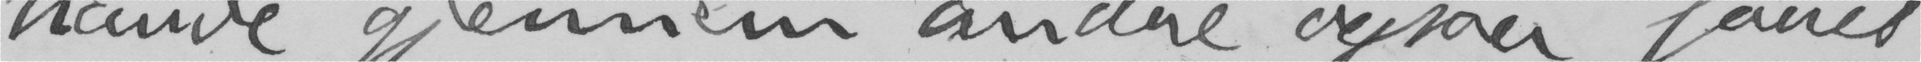havde gjennem andre ogsaa faaet
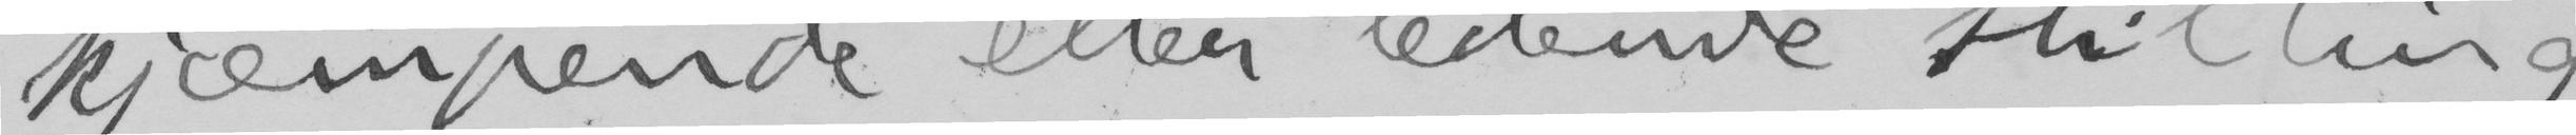kjæmpende eller ledende stilling.
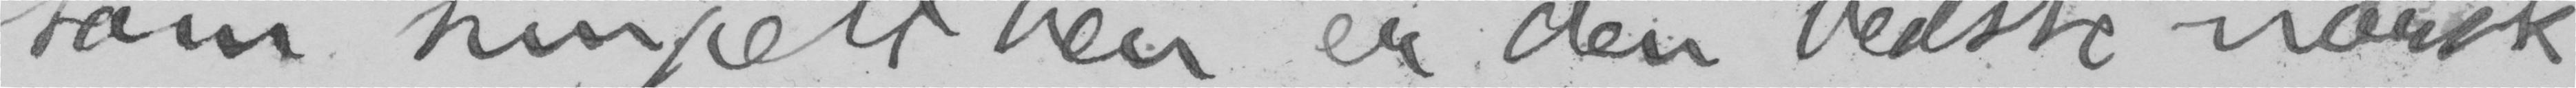som simpelt hen er den bedste norsk,
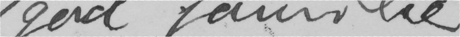god familie
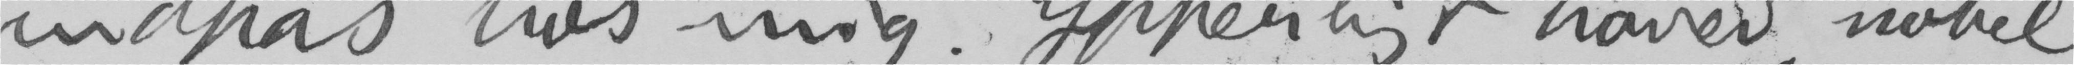indpas hos mig. Ypperligt hoved, nobel
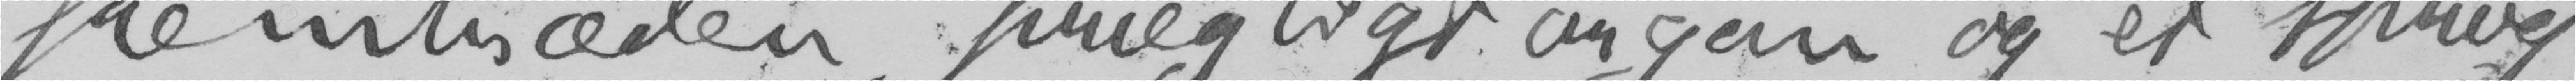fremtræden, pregtigt organ og et sprog,
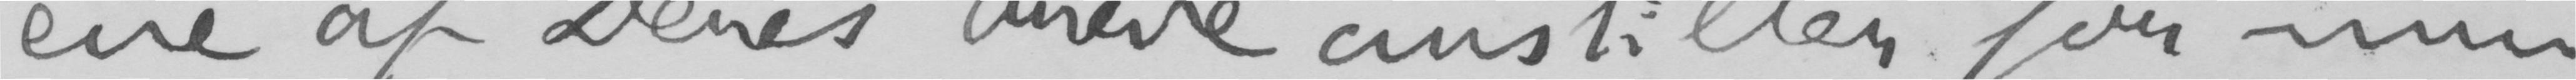ene af Deres breve anstiller for min
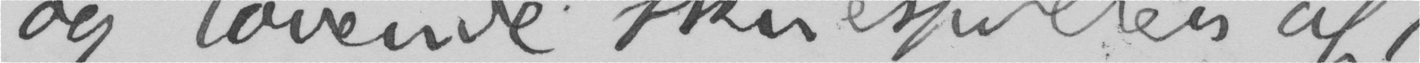og lovende skuespiller af
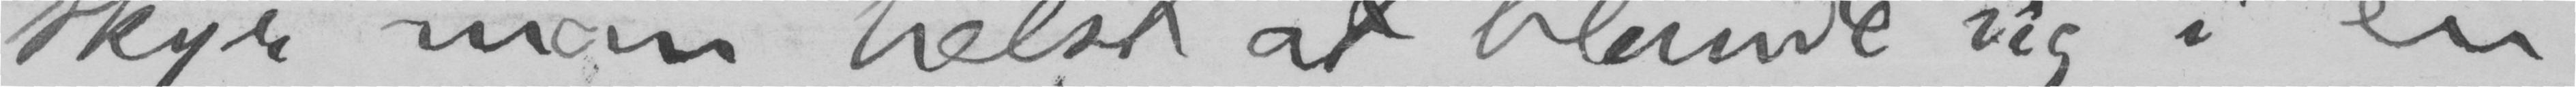skyr man helst at blande sig i en
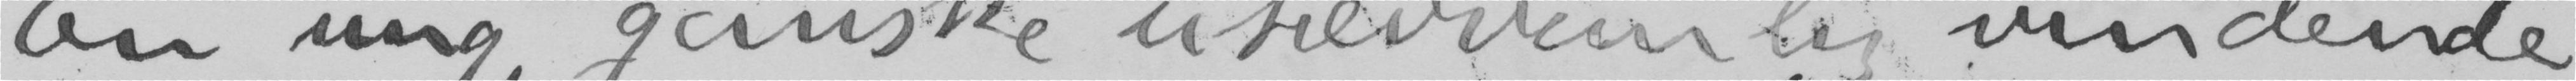En ung, ganske usædvanlig vindende
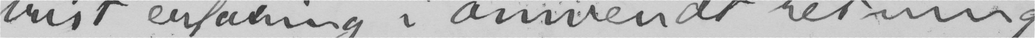trist erfaring i anvendt retning.
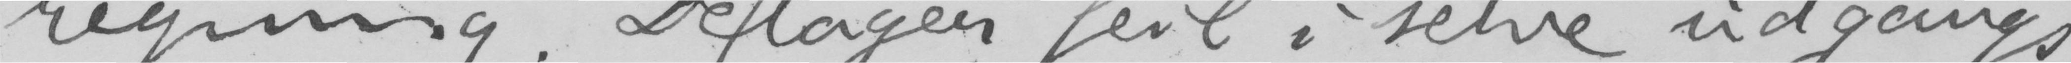regning. De tager feil i selve udgangs-
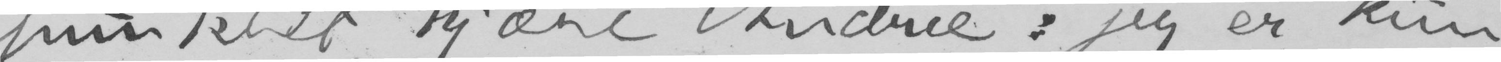punktet, kjære Andræ: jeg er kun
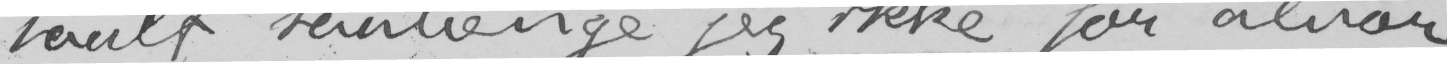taalt, saalenge jeg ikke for alvor
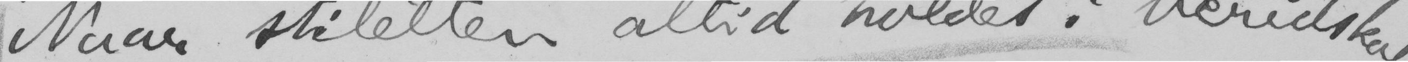Naar stiletten altid holdes i beredskab,
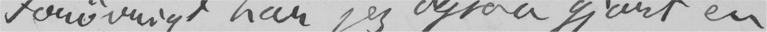Forøvrigt har jeg ogsaa gjort en
